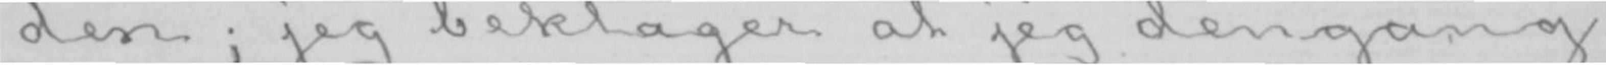den; jeg beklager at jeg dengang
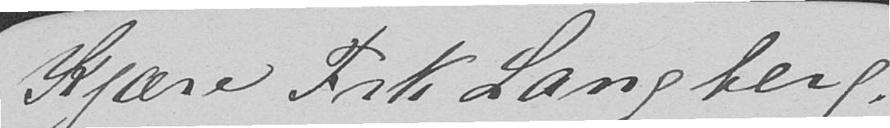Kjære Frk Langberg.
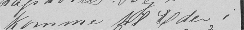komme til Eder i
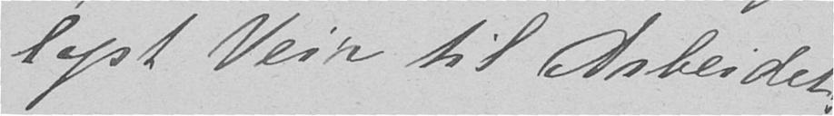lyst Veir til Arbeidet.
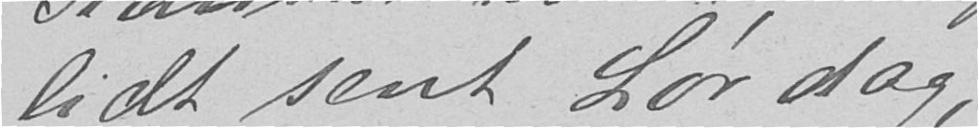lidt sent Lørdag;
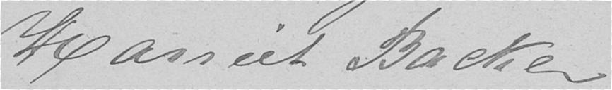Harriet Backer
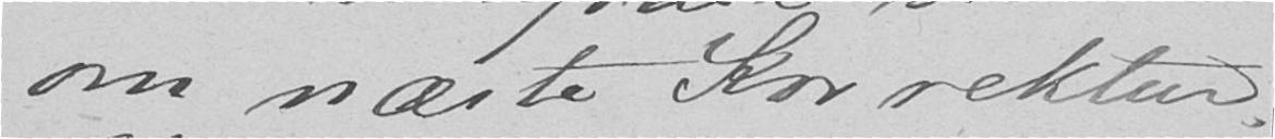om næste Korrektur.
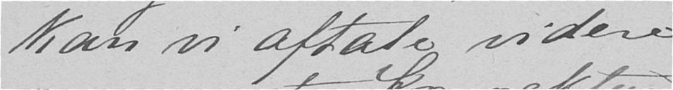kan vi aftale videre
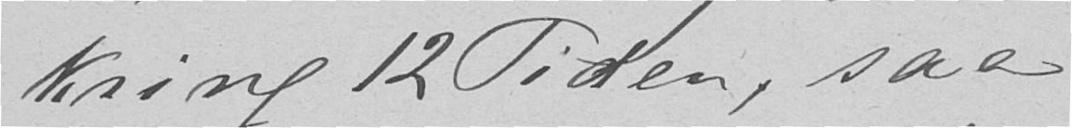kring 12 Tiden, saa
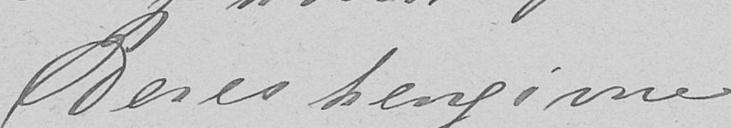Deres hengivne
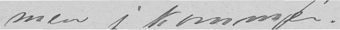men jeg kommer.
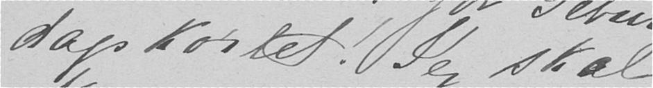dagskortet! Jeg skal
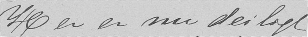Her er nu deiligt
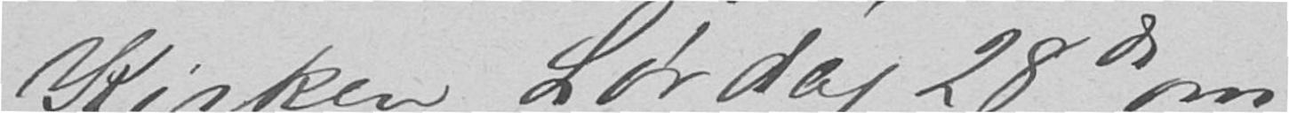Kirken Lørdag 28de om-
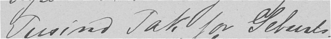Tusind Tak for Geburts-
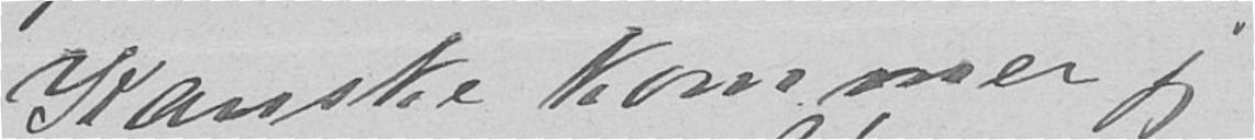Kanske kommer jeg
